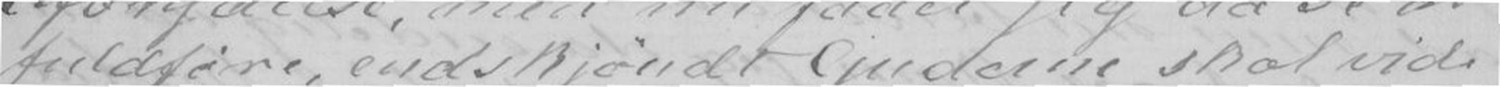fuldføre, endskjøndt Guderne skal vide
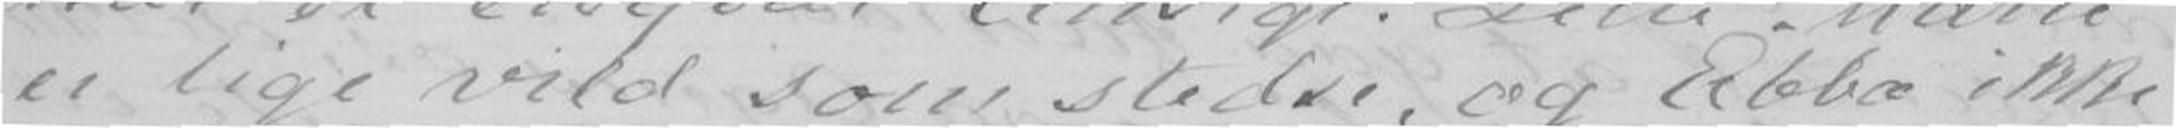er lige vild som stedse, og Ebba ikke
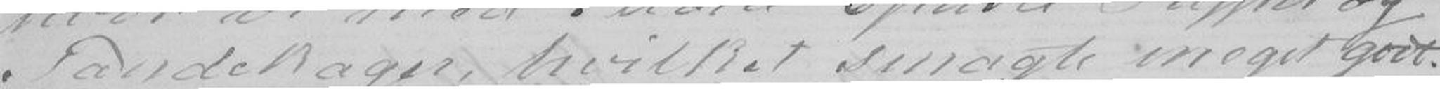Pandekager, hvilket smagte meget godt.
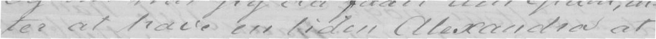ter at have en liden Alexandra at
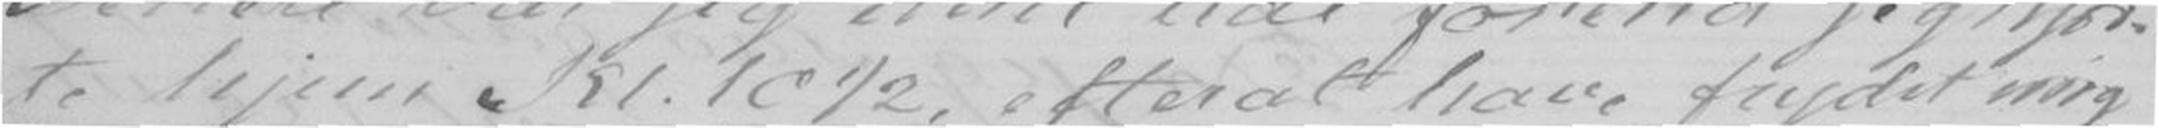te hjem Kl. 10½, efterat have frydet mig
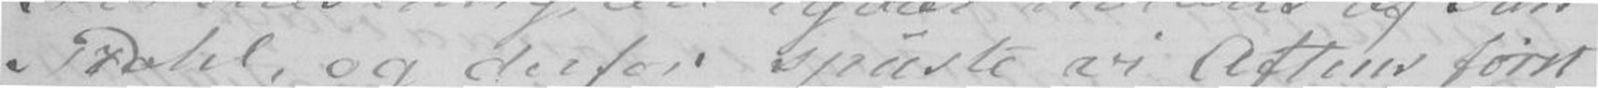Prahl, og derfor spiiste vi Aftens først
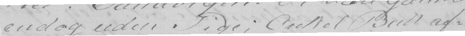endog uden Pige; Onkel Bull af-
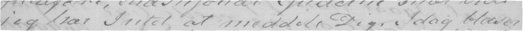jeg har Intet at meddele Dig. Idag blæser
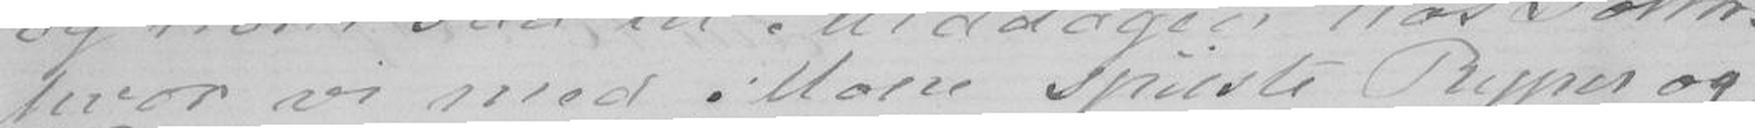hvor vi med Mone spiiste Ryper og
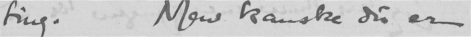ting. Men kanske du er
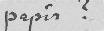papir?
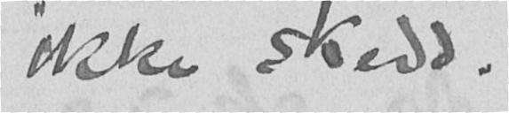ikke skedd.
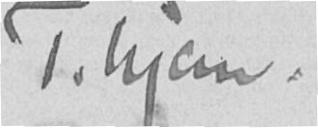T. hjem.
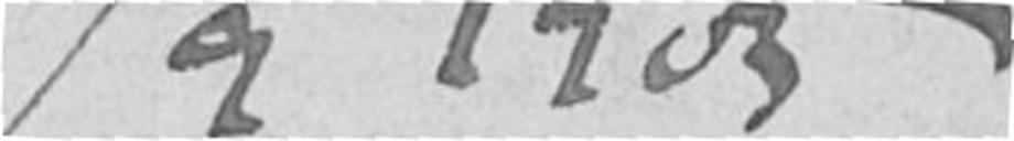/9 1905
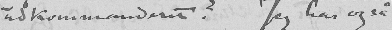udkommanderet? Jeg har også
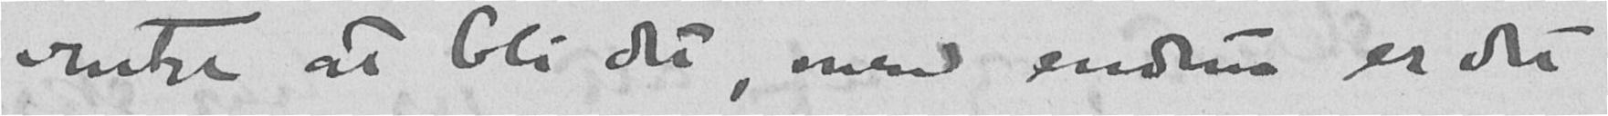ventet at bli det, men endnu er det
